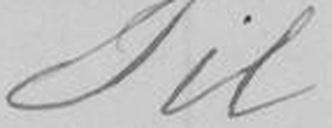Til
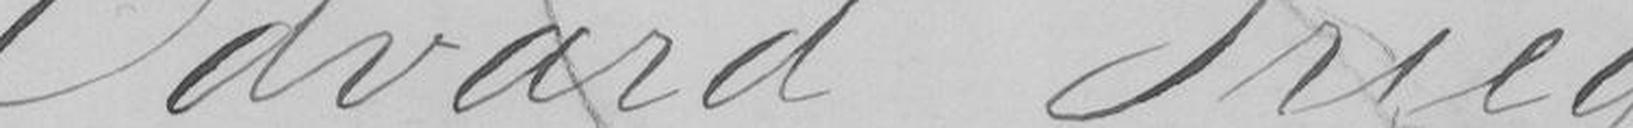Edvard Grieg
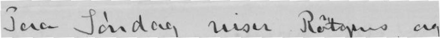Paa Søndag viser Rötgens, og
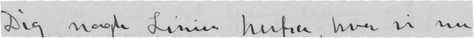Dig nogle Linier herfra, hvor vi nu
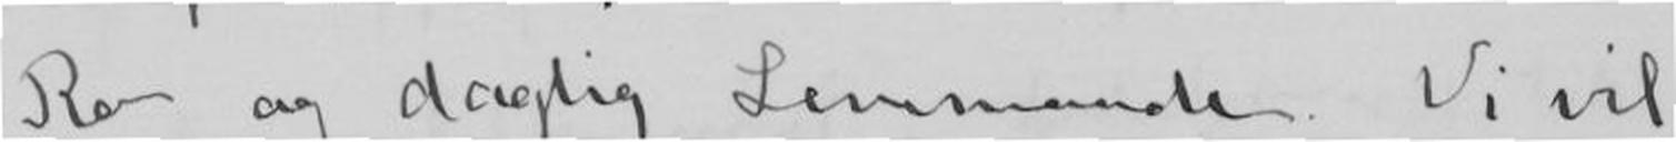Ro og daglig Levemaade. Vi vil
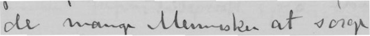de mange Mennesker at søge
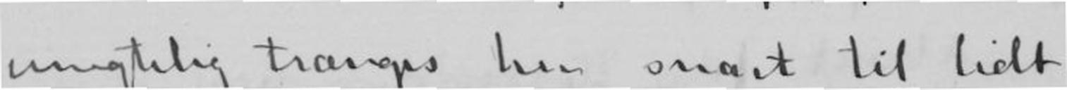megtelig trænges her snart til lidt
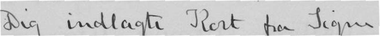Dig indlagte Kort fra Signe
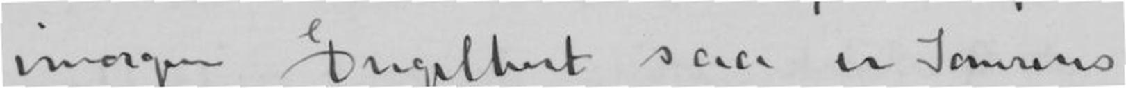imorgen Engelhart saa er Somrens
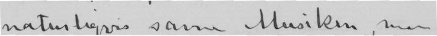naturligvis som Musiken, men
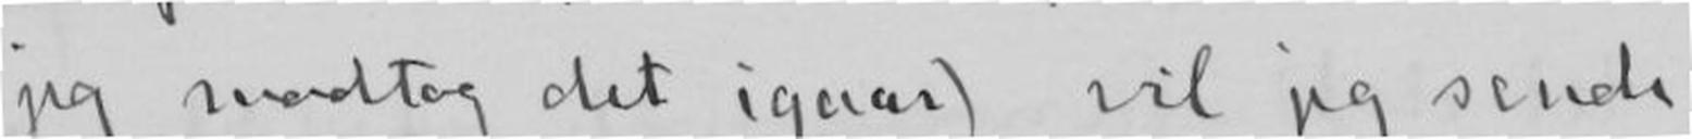(jeg modtog det igaar) vil jeg sende
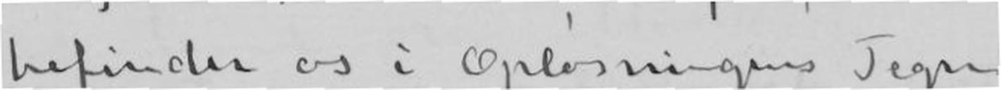befinder os i Opløsningens Tegn
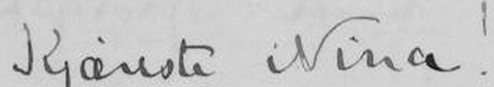Kjæreste Nina!
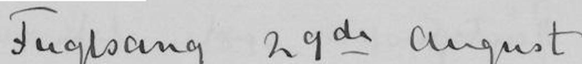Fuglsang29de August
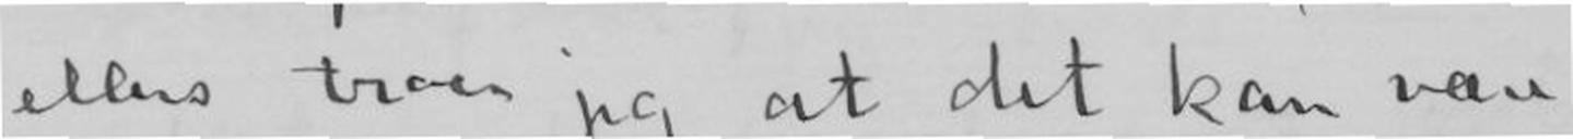ellers troer jeg at det kan være
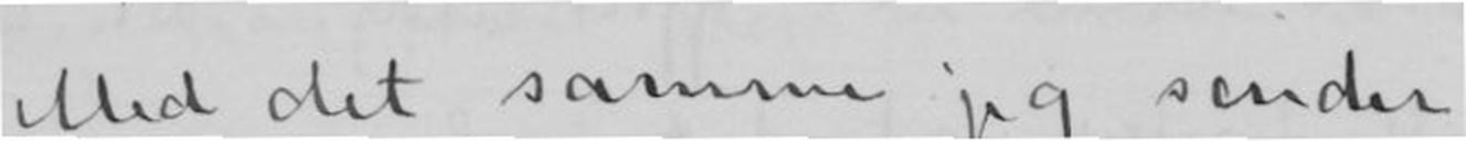Med det samme jeg sender
